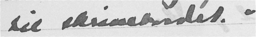til skrivebordet."
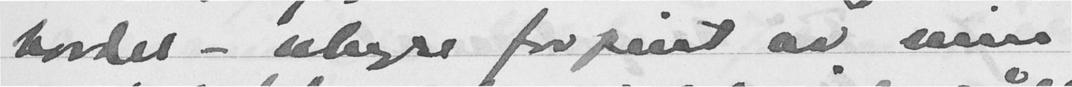bordet og uhyre forpint av min
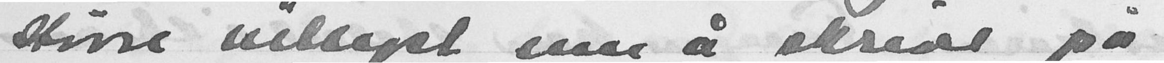større vellyst enn å skrive på
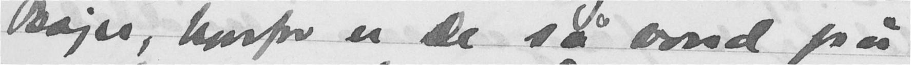Bojer, hvorfor er De så vond på
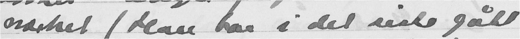nærhet (Han har i det siste gått
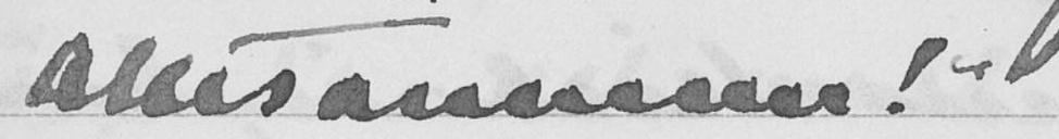allesammen!"
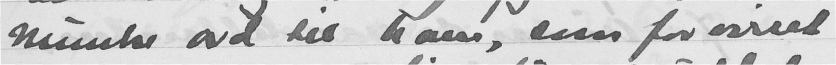muntre ord til ham, som forvirret
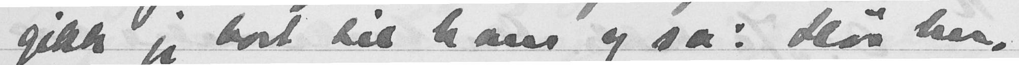gikk jeg bort til han og sa: Hør her,
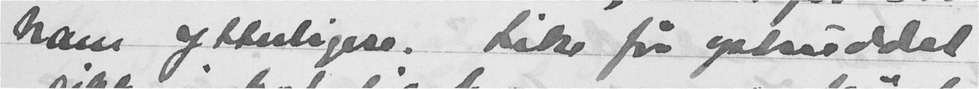ham ytterligere. Like før opbruddet
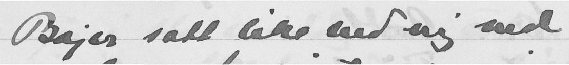Bojer satt like ved mig ved
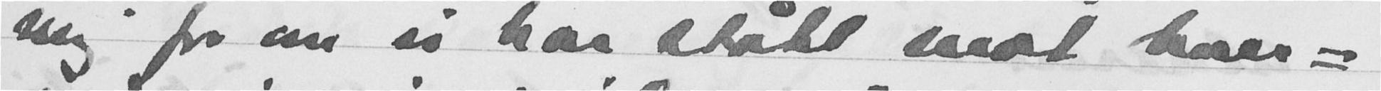mig for om vi har stått mot hver-
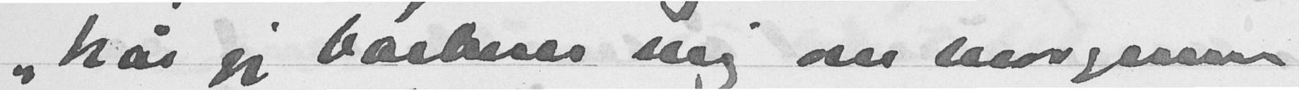"Når jeg barberer mig om morgenen
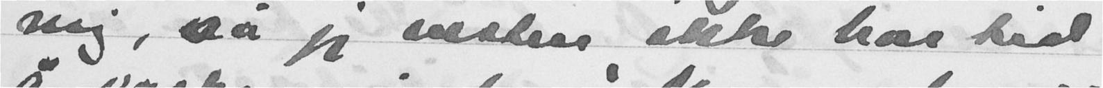mig, så jeg nesten ikke har tid
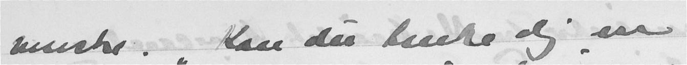veste. "Kan du tenke dig en
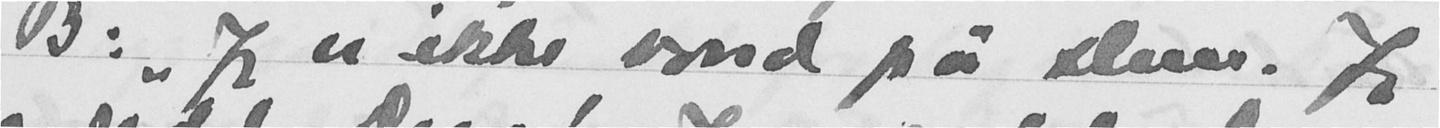B. Jeg er ikke vond på dem. Jeg
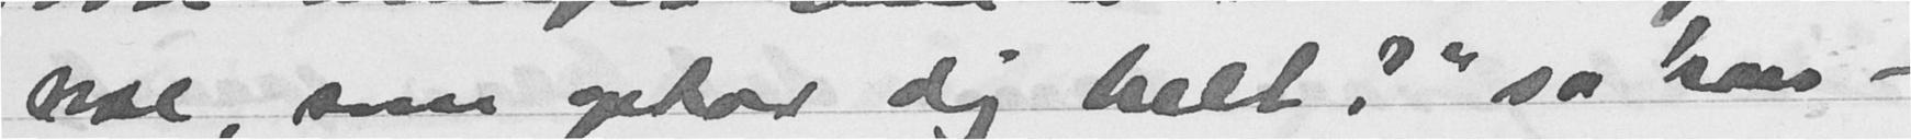noe, som optar dag helt?" sa han -
